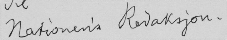Nationens Redaksjon.
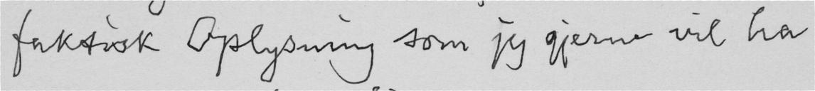faktisk Oplysning som jeg gjerne vil ha
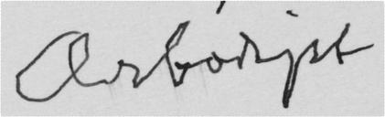Ærbødigst
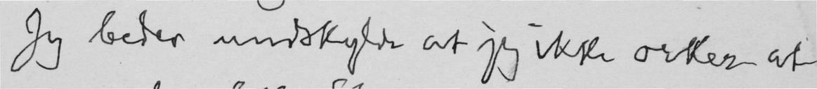Jeg beder undskylde at jeg ikke orker at
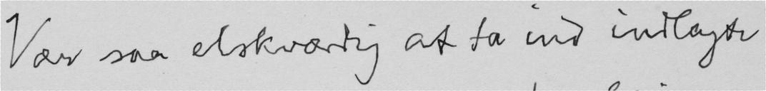Vær saa elskværdig at ta ind indlagte
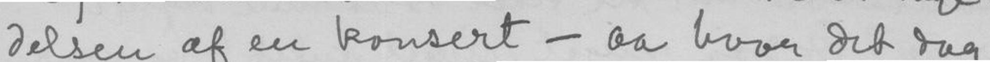delsen af en konsert - aa hvor det dog
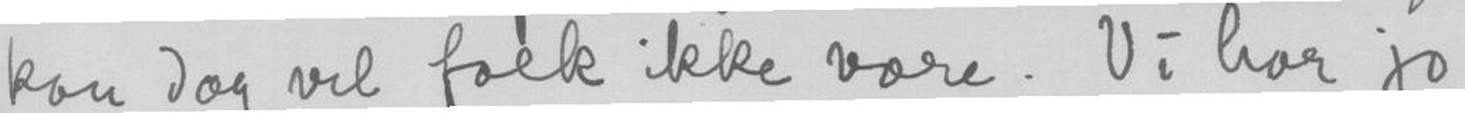kan dog vel folk ikke være. Vi har jo
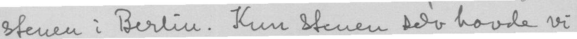stenen i Berlin. Kun stenen selv havde vi
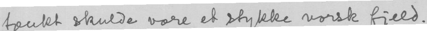tænkt skulde være et stykke norsk fjeld.
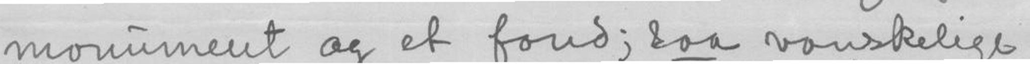monument og et fond; saa vanskelige
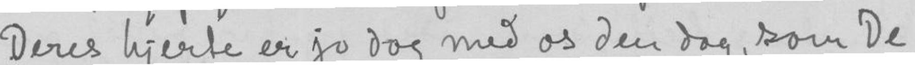Deres hjerte er jo dog med os den dag, som De
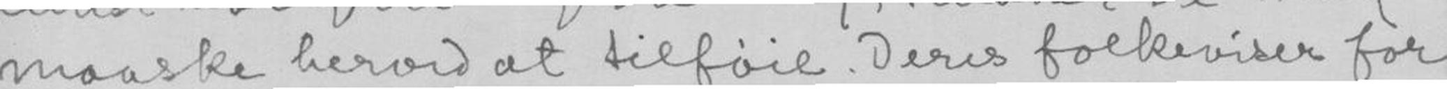maaske herved at tilføie. Deres folkeviser for
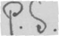P.S.
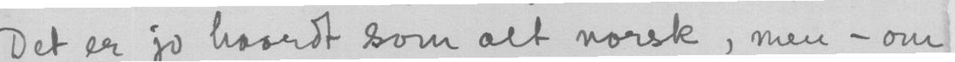Det er jo haardt som alt norsk, men - om
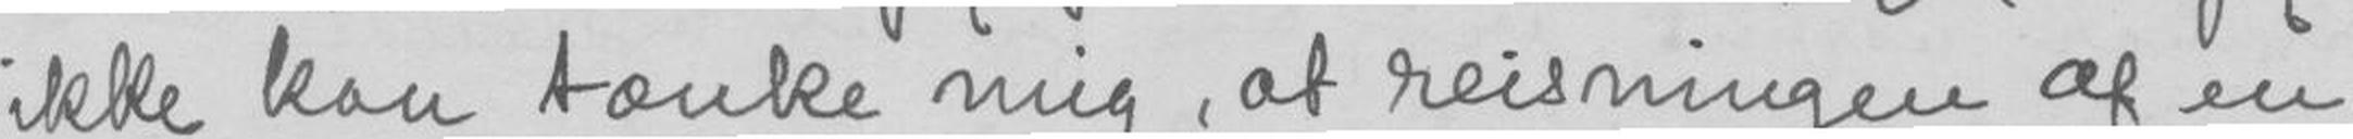ikke kan tænke mig, at reisningen af en
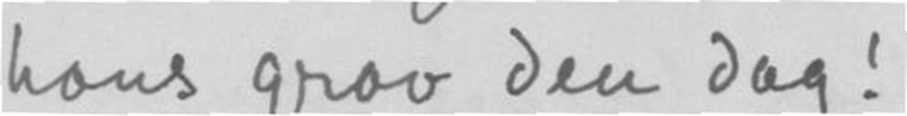hans grav den dag!
